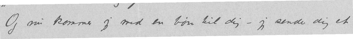Og nu kommer jeg med en bøn til dig - jeg sender dig et
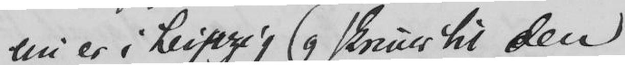nu er i Leipzig og skriver til den
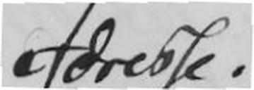Adresse.
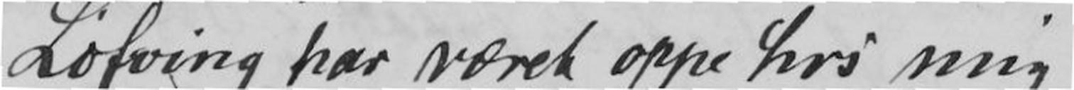Løfving har været oppe hos mig
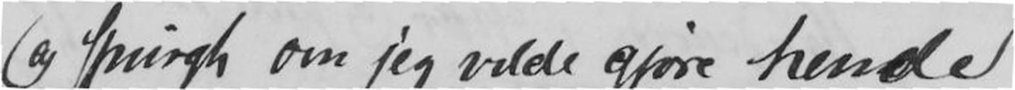og spurgt om jeg vilde gjøre hende
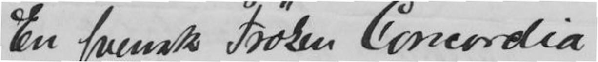En svensk Frøken Concordia
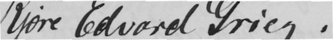Kjære Edvard Grieg!
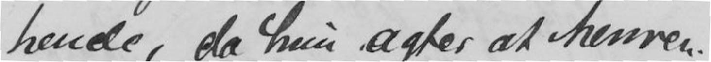hende, da hun agter at henven-
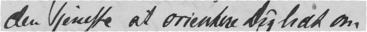den Tjeneste at orientere Dig lidt om
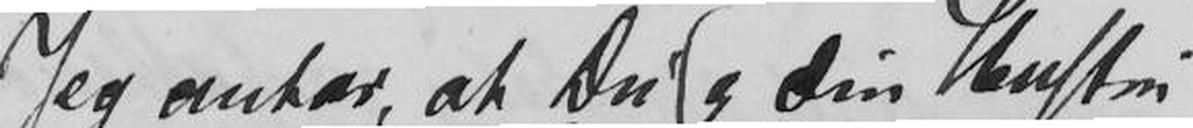Jeg antar, at Du og din Hustru
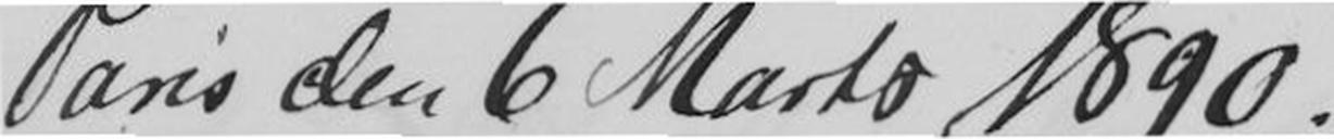Paris den 6 Marts 1890.
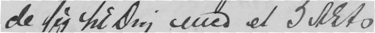de sig til Dig med et 5 Akts
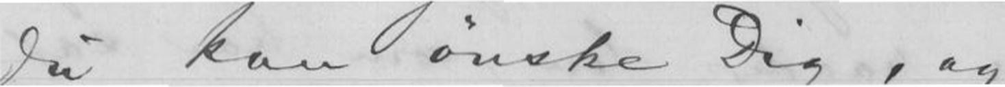Du kan ønske Dig, og
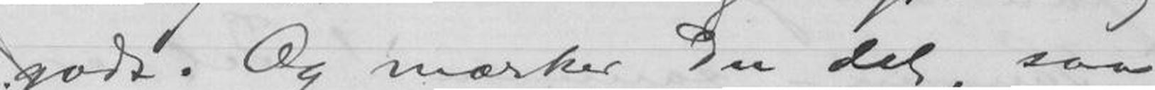godt. Og mærker Du det, saa
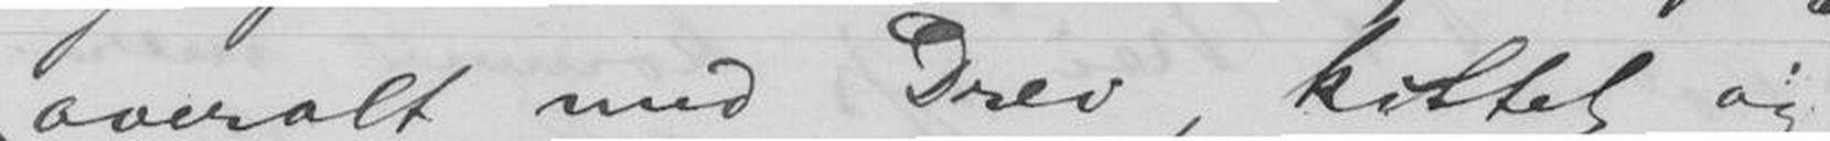overalt med Drev, kittet og
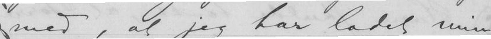med, at jeg har ladet min
